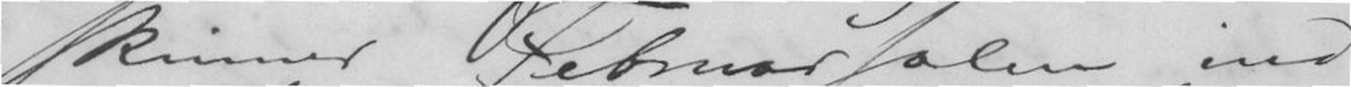skinner Februarsolen ind
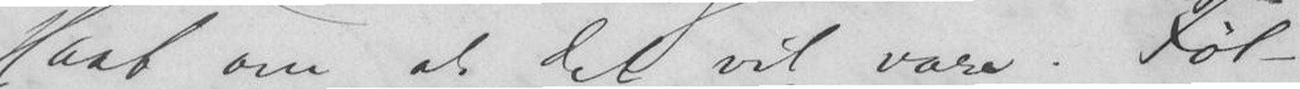Haab om at det vil vare. Føl-
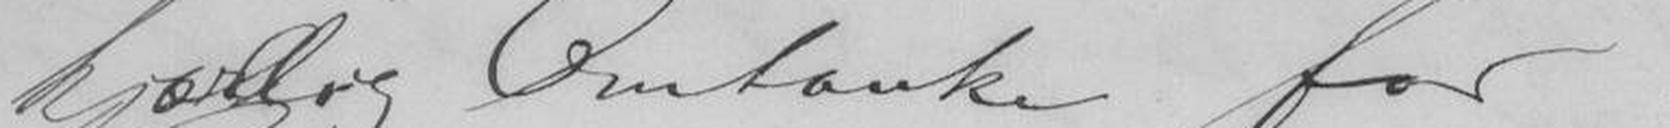kjærlig Omtanke for
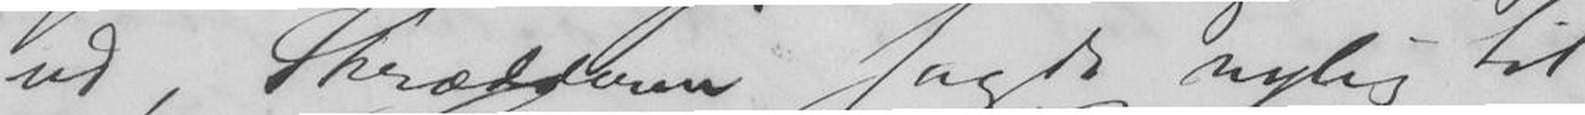ud, Skrædderen sagde nylig til
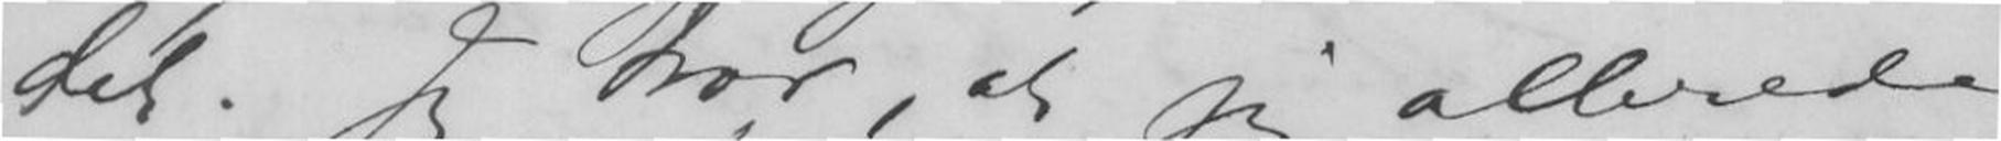det. Jeg tror, at jeg allerede
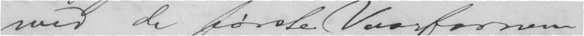med de første Vaarfornem-
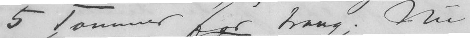5 Tommer for trang. Nu
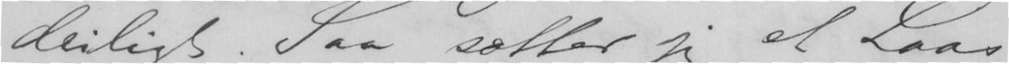deiligt. Saa sætter jeg et Laas
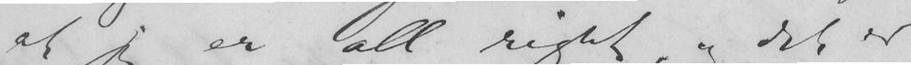at jeg er all right, og det er
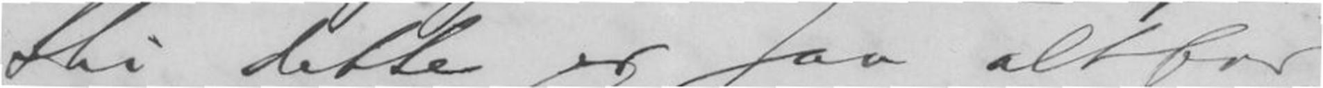thi dette er saa altfor
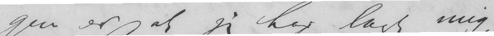gen er at jeg har lagt mig
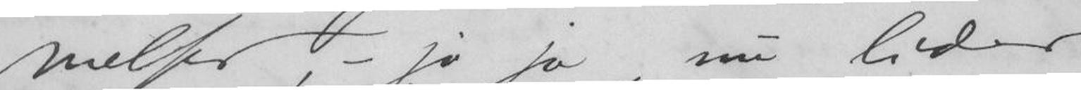melser, - ja ja, nu lider
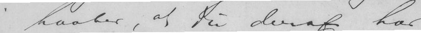jeg haaber, at du deraf har
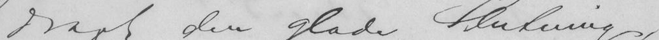draget den glade Slutning,
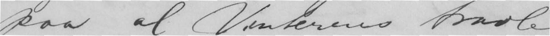paa al Vinterens travle
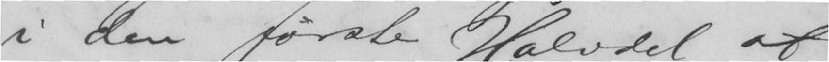i den første Halvdel af
